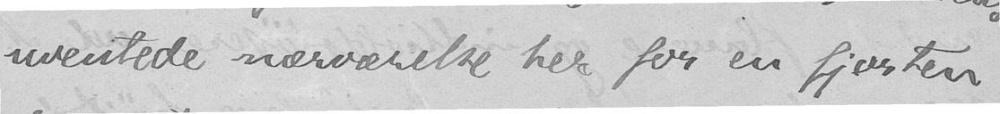uventede nærværelse her for en fjorten
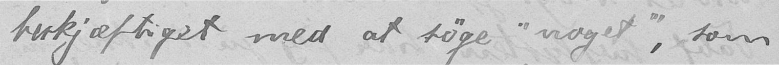beskjæftiget med at søge "noget", som
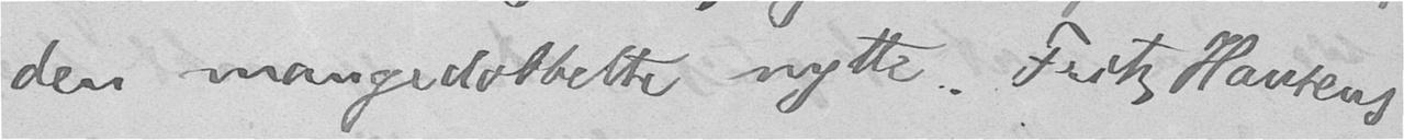den mangedobbelte nytte. Fritz Hansens
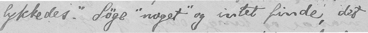lykkedes." Søge "noget" og intet finde, det
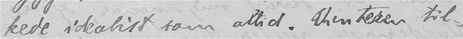kede idealist som altid. Vinteren til-
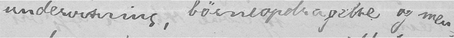undervisning, børneopdragelse og men-
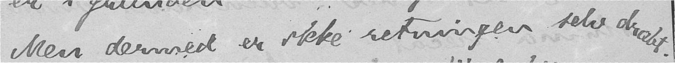Men dermed er ikke retningen selv dræbt.,
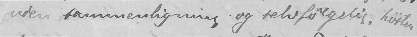uden sammenligning og selvfølgelig, høster
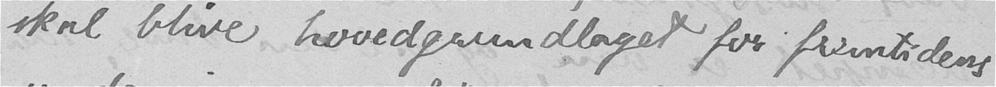skal blive hovedgrundlaget for fremtidens
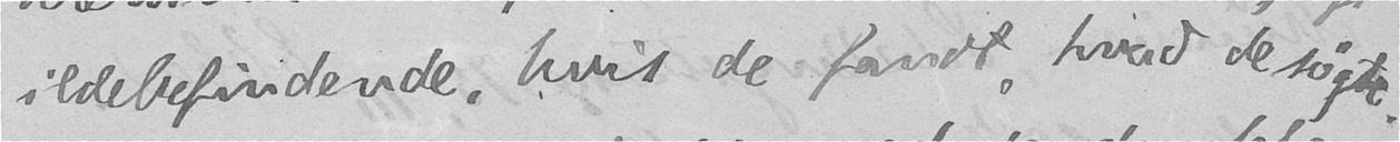ildebefindende, hvis de fandt, hvad de søgte.
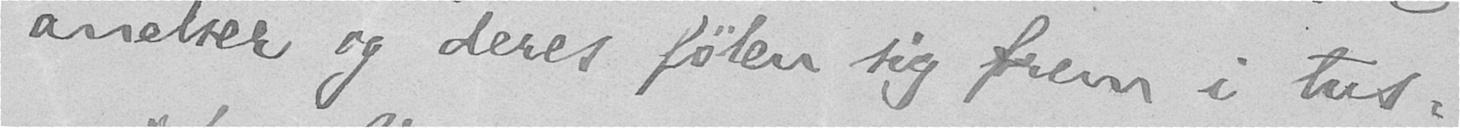anelser og deres følen sig frem i tus-
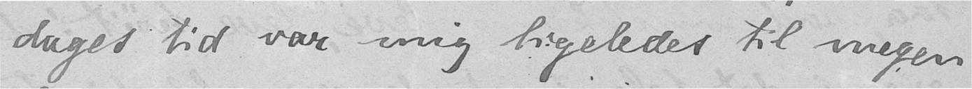dages tid var mig ligeledes til megen
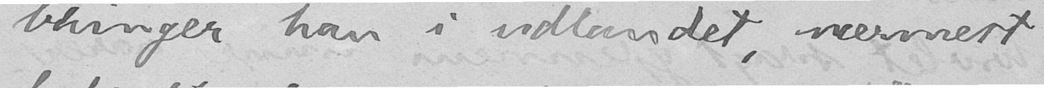bringer han i udlandet, nærmest
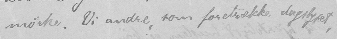mørke. Vi andre, som foretrække dagslyset,
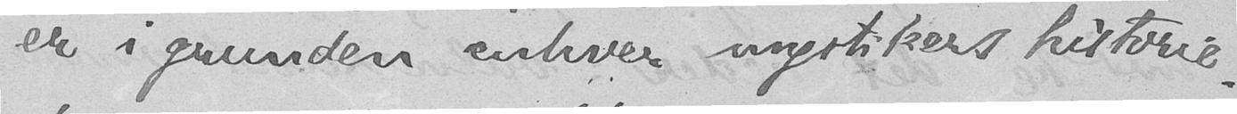er i grunden enhver mystikers historie.
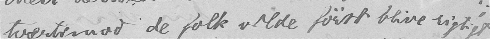tværtimod de folk vilde først blive rigtigt
